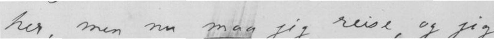her, men nu maa jeg reise, og jeg
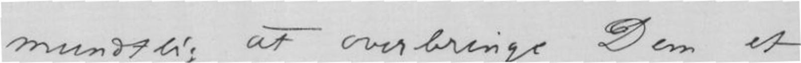mundtlig at overbringe Dem et
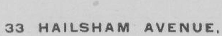33 HAILSHAM AVENUE.
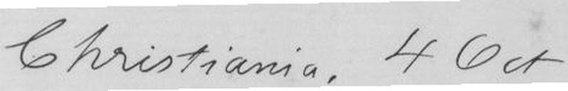Christiania. 4 Oct
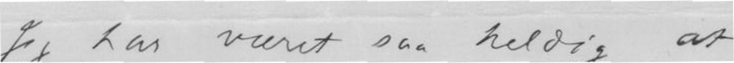Jeg har været saa heldig at
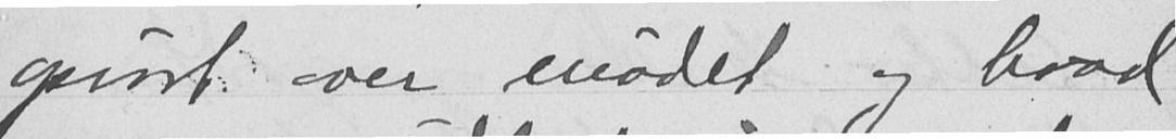oprørt over mødet og hvad
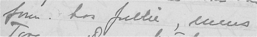forn. hos Julie, mens
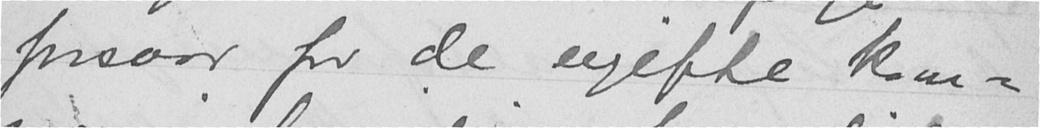forsvar for de ugifte kom-
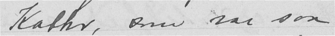Katti, som var saa
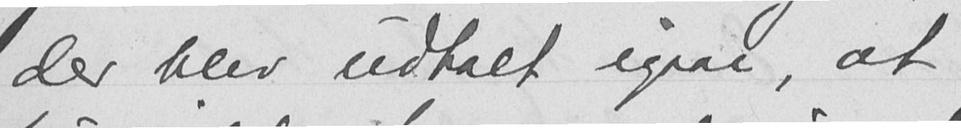der blev udtalt igaar, at
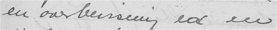en overbevisning er en
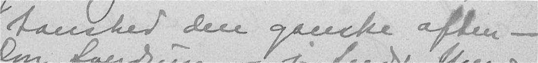taushed den ganske aften -
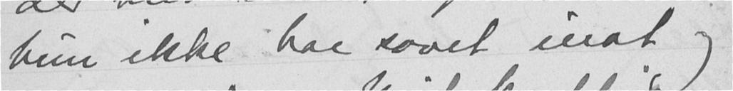hun ikke har sovet inat og
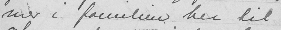mer i familien her til
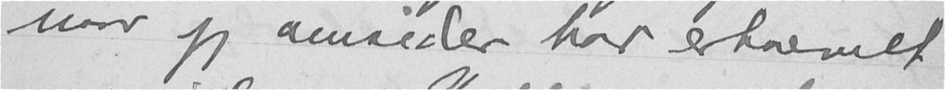naar jeg omsider har erhvervet
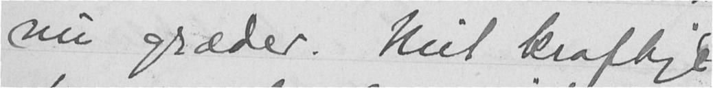nu græder. Mit kraftige
